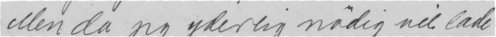Men da jeg yderlig nødig vil lade
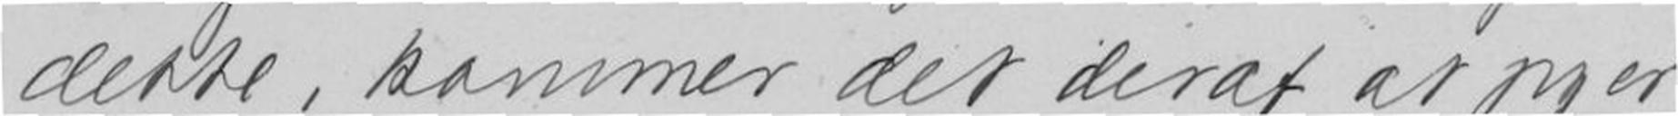dette, kommer det deraf at jeg er
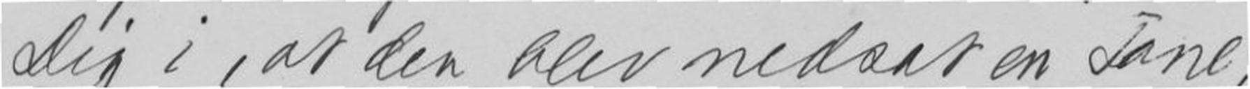Dig i, at der blev nedsat en Tone,
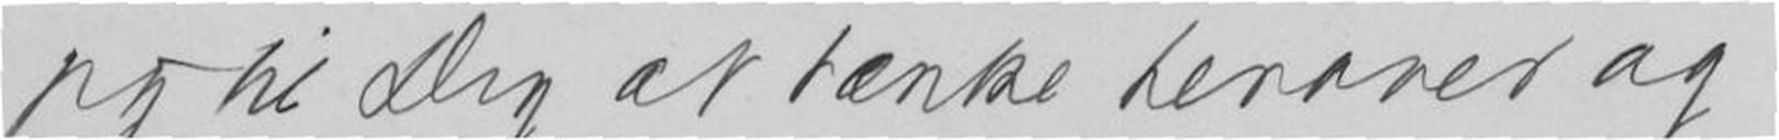jeg til Dig at tænke henover og
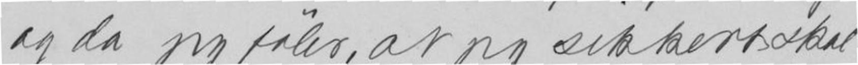og da jeg føler, at jeg sikkert skal
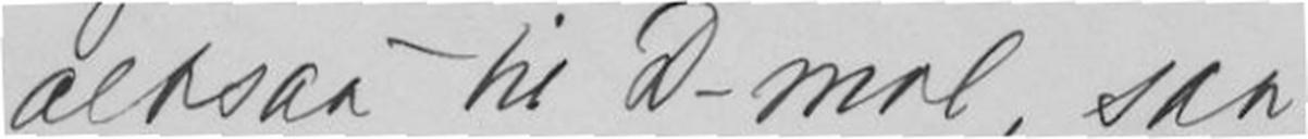altsaa til D-mol, saa
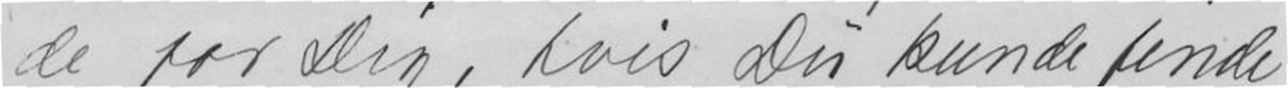de for Dig, hvis Du kunde finde
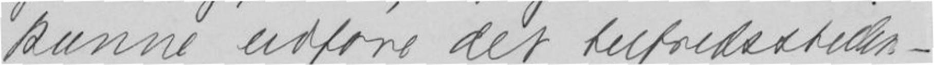kunne udføre det tilfredsstillen-
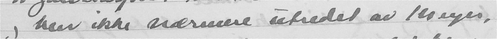og blir ikke nærmere utredet av Meyer,
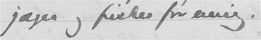jæger og fisker forening.
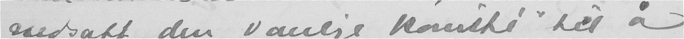nedsatt den vanlige "komité" til å
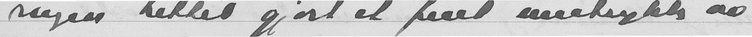ringen hittil gjort et fint inntrykk av
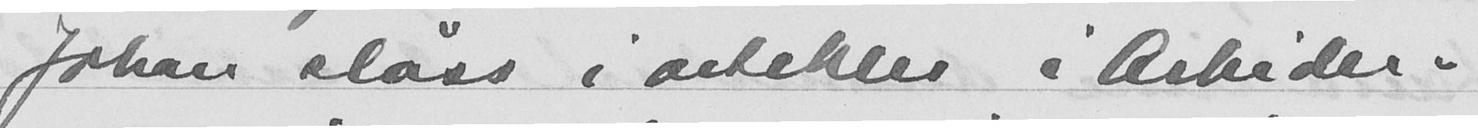Johan slåss i artikler i Arbeider-
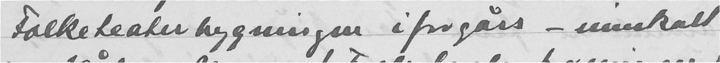Folketeaterbygningen iforgårs - innkalt
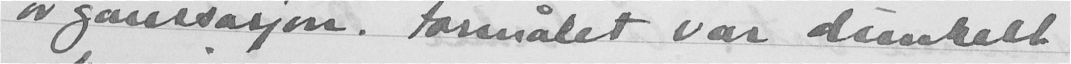organisasjon. Formålet var dunkelt
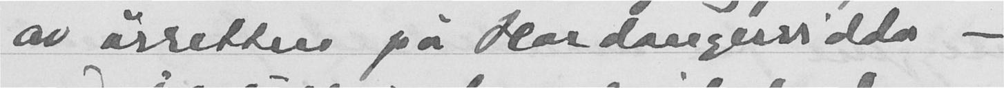av ørretten pa Hardangervidda -
